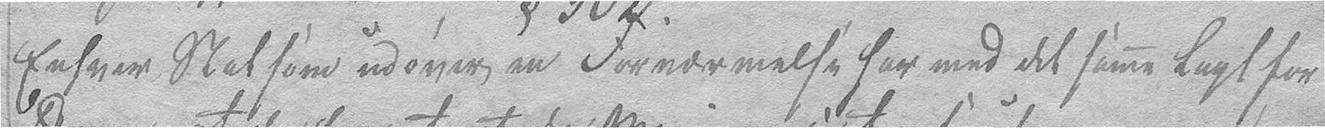Enhver Stat som udøver en Fornærmelse har med det samme lagt for
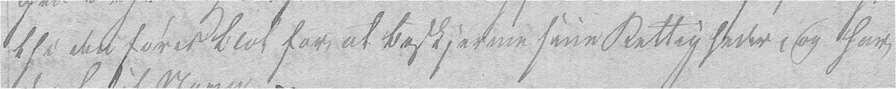thi det føres blot for at beskjærme sine Rettigheder, og har
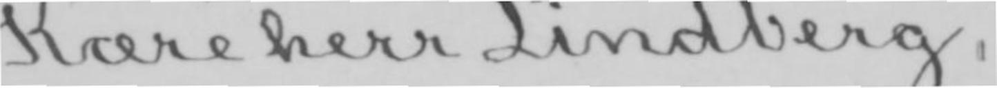Kære herr Lindberg,
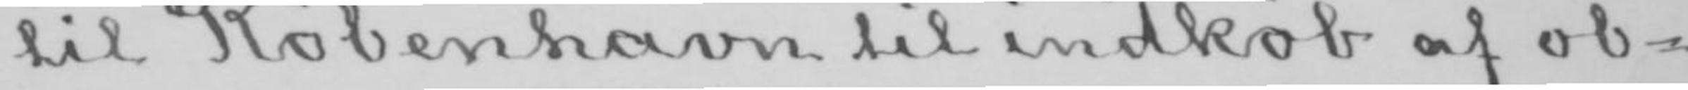til København til indkøb af ob-
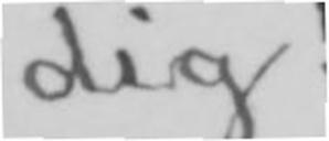dig!
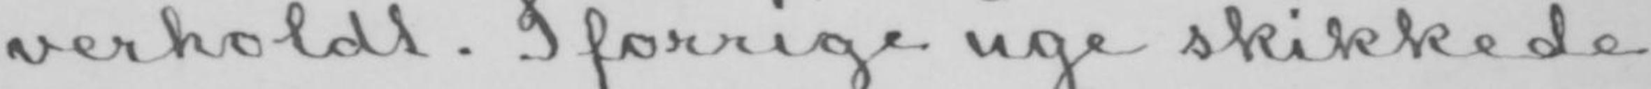verholdt. I forrige uge skikkede
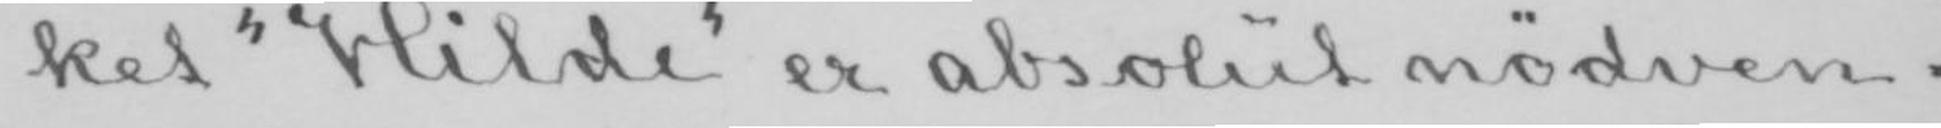ket «Hilde» er absolut nødven-
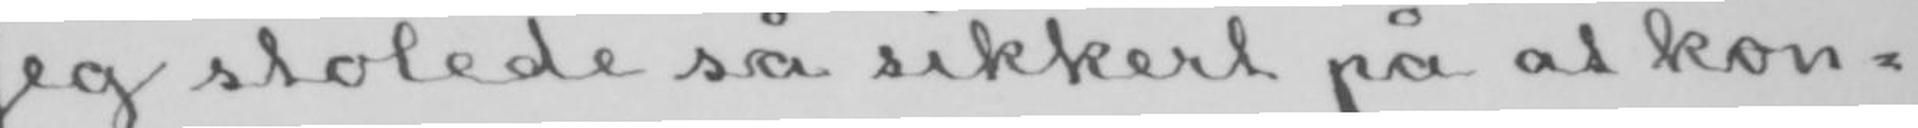Jeg stolede så sikkert på at kon-
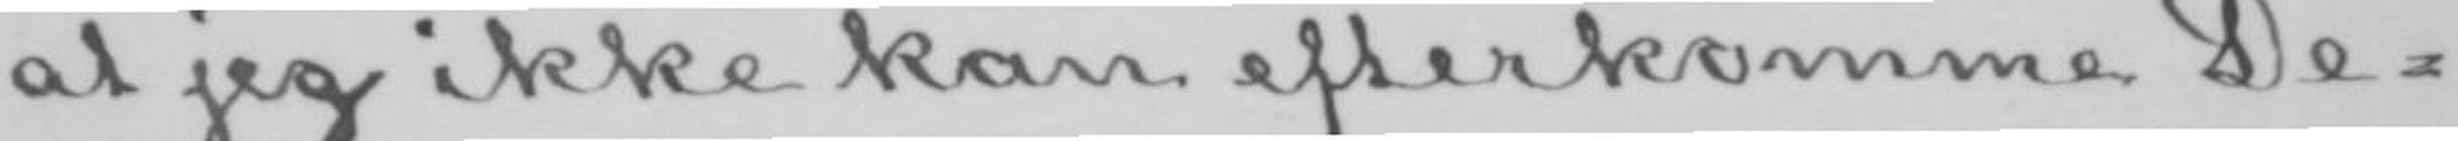at jeg ikke kan efterkomme De-
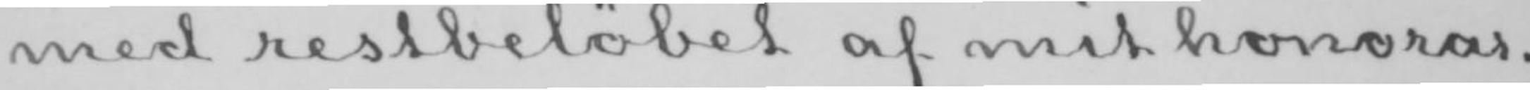med restbeløbet af mit honorar.
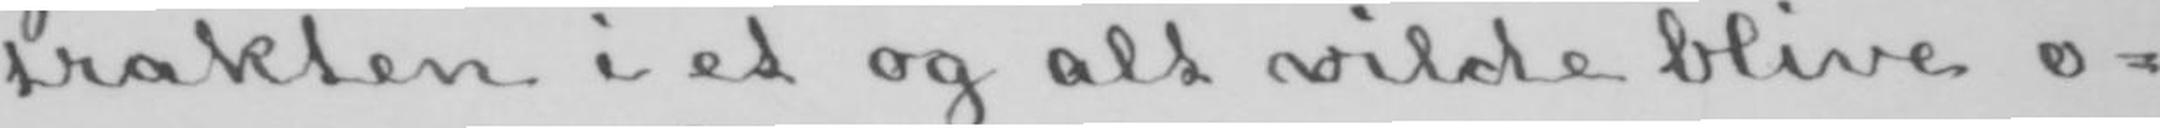trakten i et og alt vilde blive o-
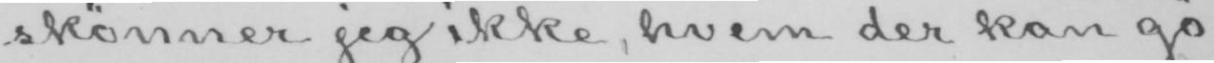skønner jeg ikke, hvem der kan gø-
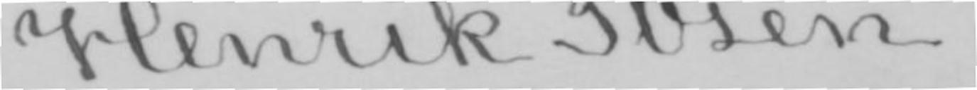Henrik Ibsen.
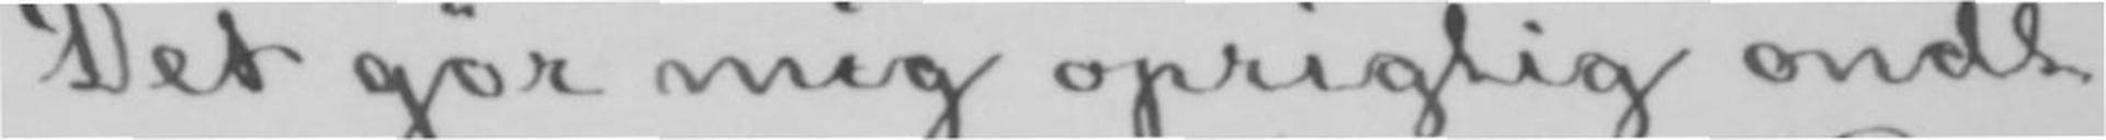Det gør mig oprigtig ondt
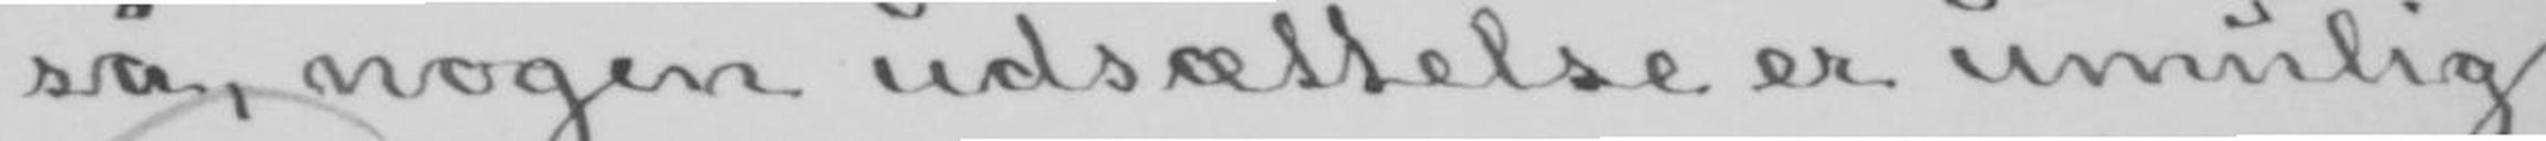så, nogen udsættelse er umulig.
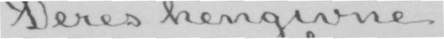Deres hengivne
